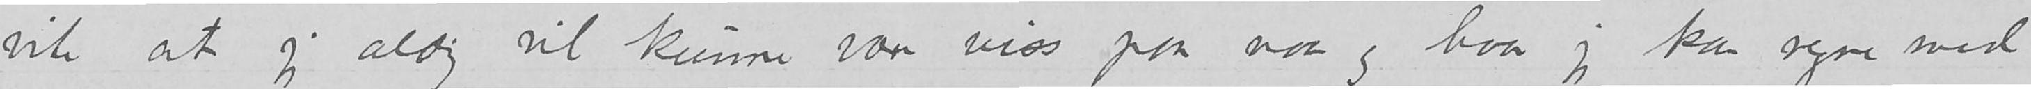jeg aldrig vil kunne være viss paa naar og hvor jeg kan regne  med
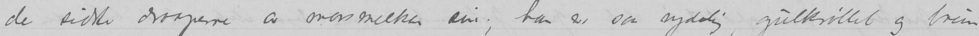de sidste draaperne av morsmelken sin;  han er saa nydelig, gulkrøllet og brun
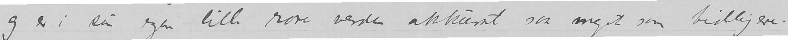og er i sin egen lille rare verden akkurat saa meget som tidligere.
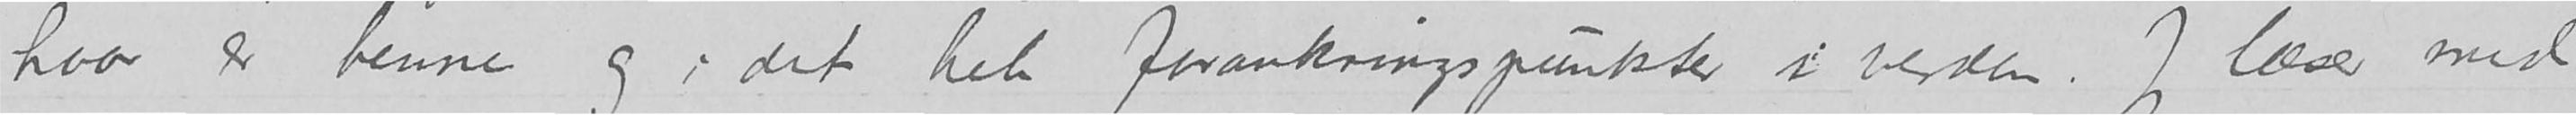hvor er henne og i det hele forankringspunkter i verden.  Jeg læser med
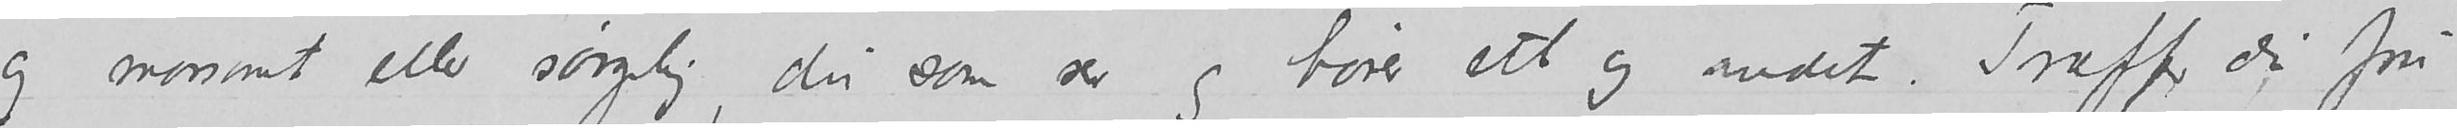og morsomt eller sørgelig, du som ser og hører ett og andet. Træffer du fru
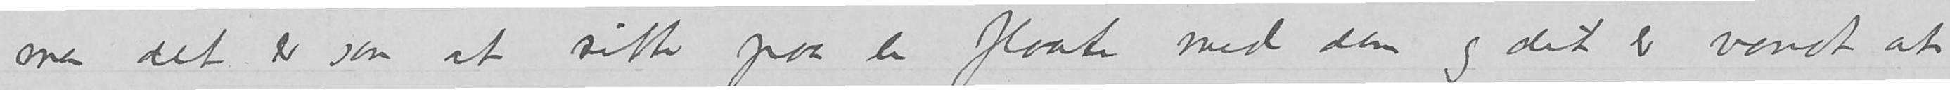men det er som at sitte paa en flaate med dem og det er vondt at vite at
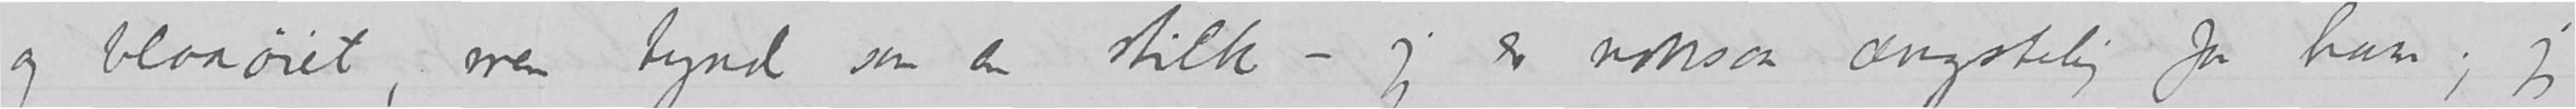og blaaøiet, men tynd som en stilk – jeg er noksaa ængstelig for ham;  jeg
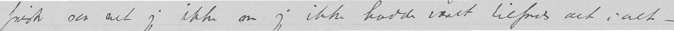frisk saa vet jeg ikke om jeg ikke havde været tilfreds alt i alt –
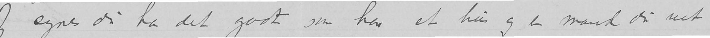Jeg synes du har det godt som har et hus og en mand du vet
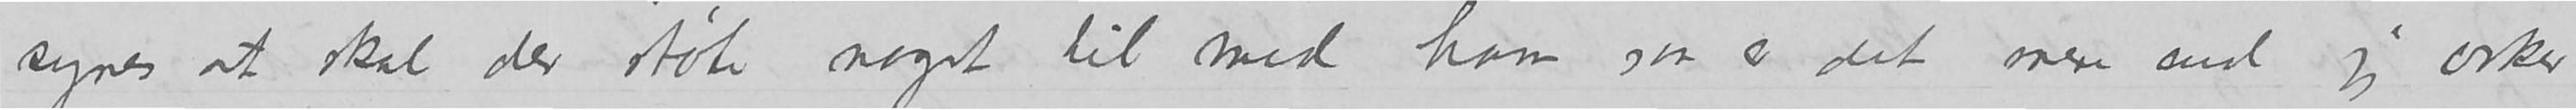synes at skal der støte noget til med ham saa er det mere end jeg orker
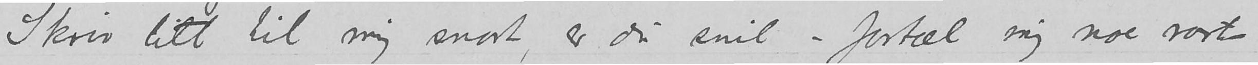Skriv litt til mig snart, er du snil – fortæl mig noe rart
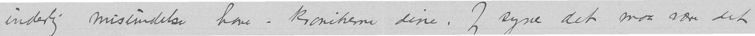inderlig misundelse have-kronikerne dine.  Jeg synes det maa være det
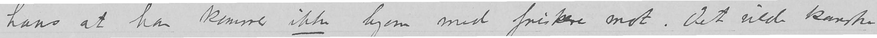hans at han kommer ikke hjem med friskere mot.  Det vilde kanske
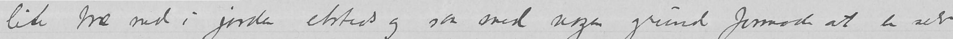kunde grave et lite træ ned i jorden etsteds og saa med nogen grund formode at en selv
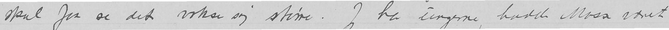skal faa se det vokse sig større.  Jeg har ungerne, hadde Mosse været
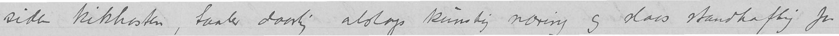siden kikhosten, taaler daarlig alslags kunstig næring og slaas/oss standhaftig for
